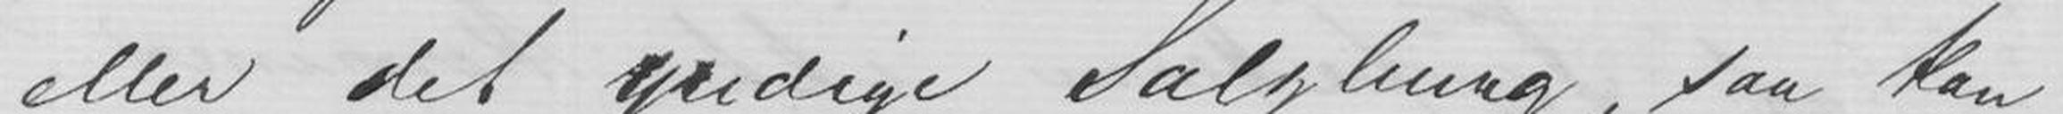eller det yndige Salzburg, saa kan
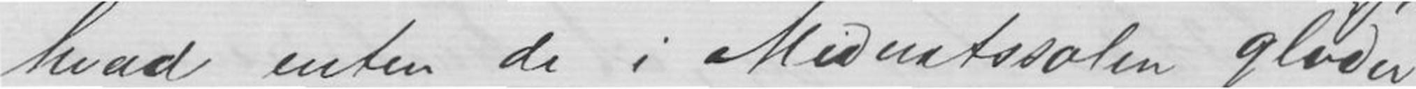hvad enten de i Midnatssolen gløder
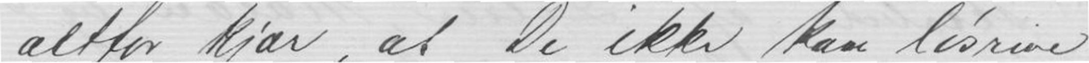altfor kjær, at De ikke kan løsrive
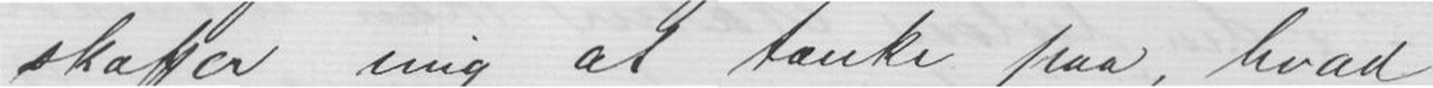skaffer mig at tænke paa, hvad
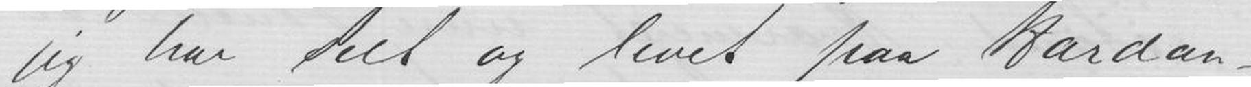jeg har seet og levet paa Hardan-
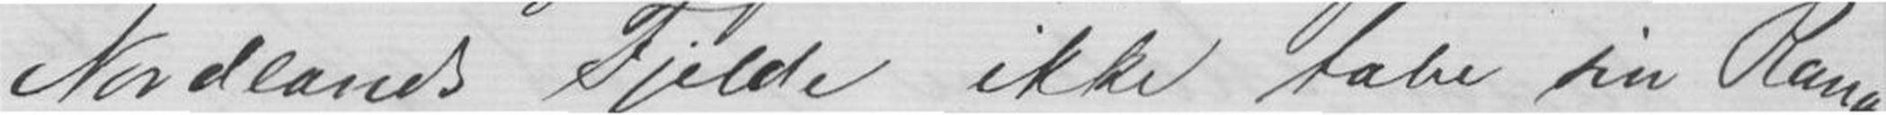Nordlands Fjelde ikke tabe sin Rang,
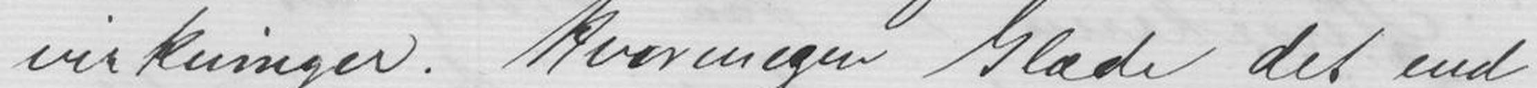virkninger. Hvormegen Glæde det end
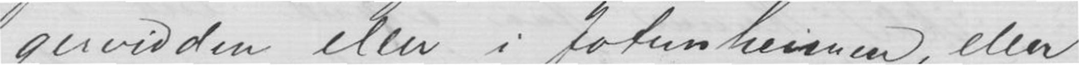gervidden eller i Jotunheimen, eller
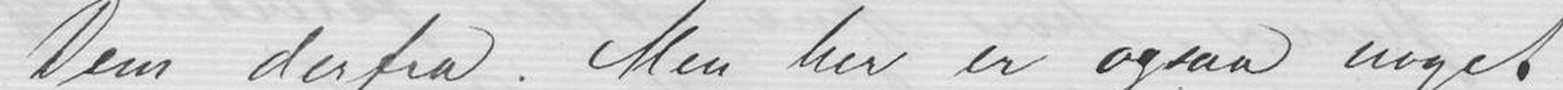Dem derfra. Men her er ogsaa noget
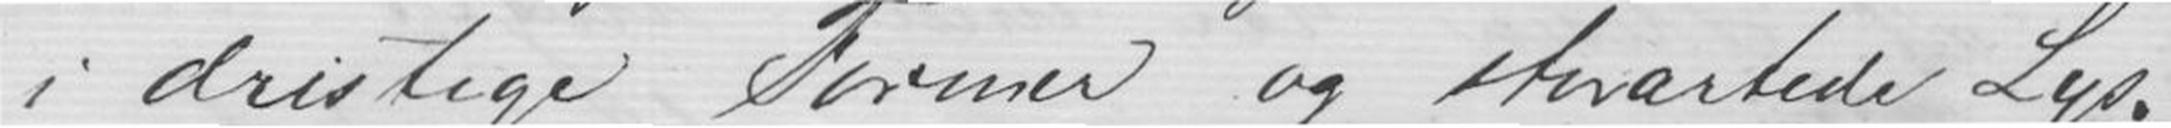i dristige Former og storartede Lys-
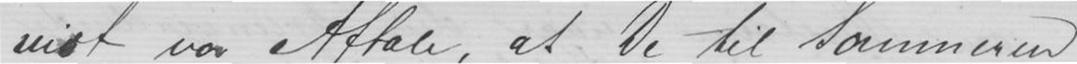vist vor Aftale, at De til Sommeren
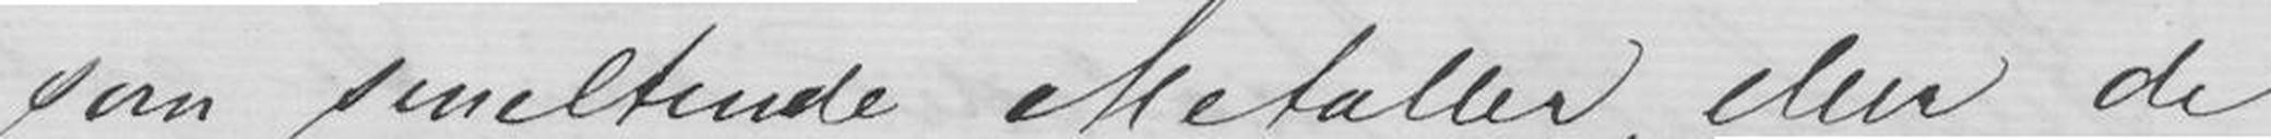som smeltende Metaller, eller de
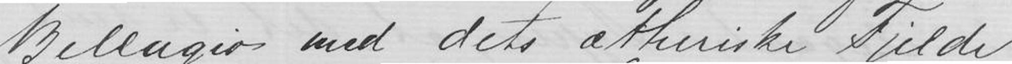Bellagio med dets atheriske Fjelde
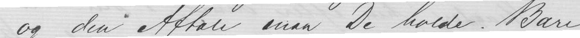og den Aftale maa De holde. Bare
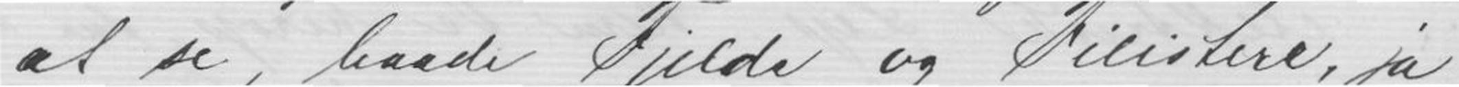at se, baade Fjelde og Filistere, ja
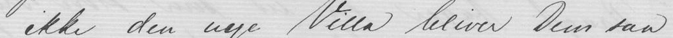ikke den nye Villa bliver Dem saa
